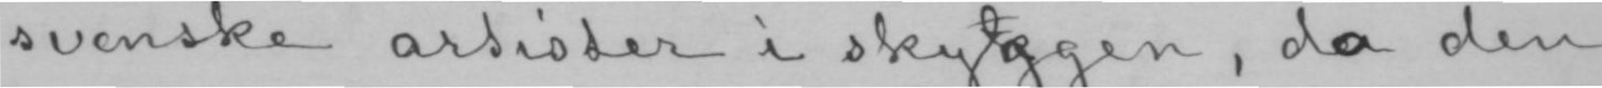svenske artister i skyggen, dea den
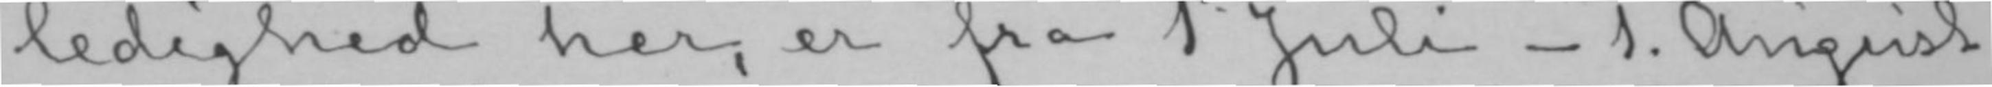ledighed her, er fra 1ste Juli- 1. August
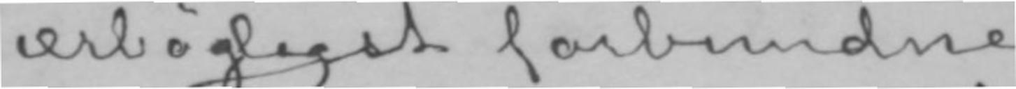ærbøgligst forbundne
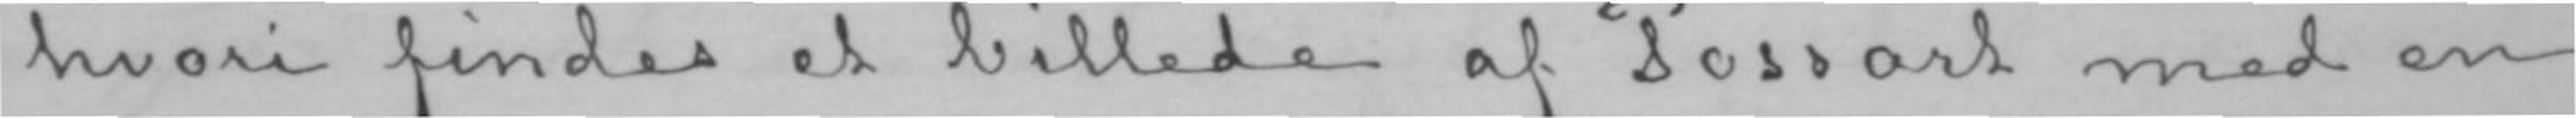hvori findes et billede af Possart med en
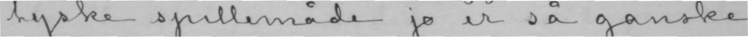tyske spillemåde jo er så ganske
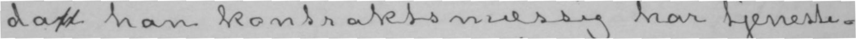dan han kontraktsmæssig har tjeneste-
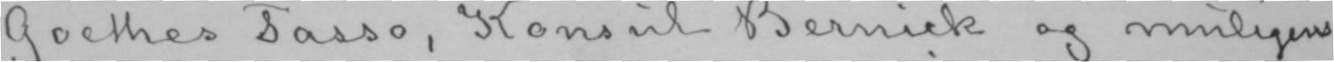Goethes Tasso, Konsul Bernick og muligens
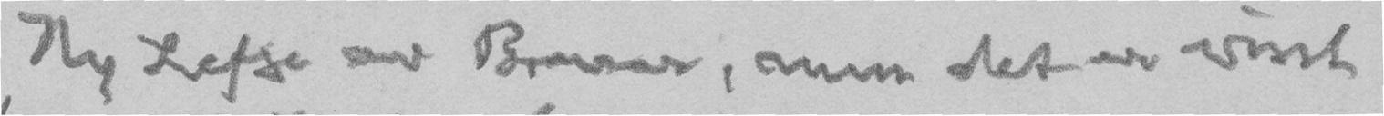Ny Lefse av Brever, men det er visst
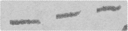- - -
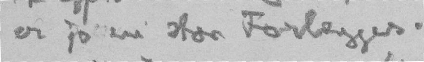er jo en stor Forlægger.
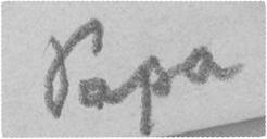Papa
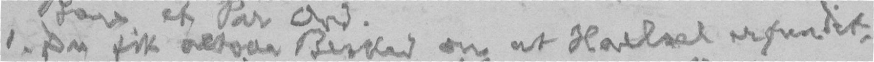1. Du fik altsaa Besked om at Haelsel er fundet,
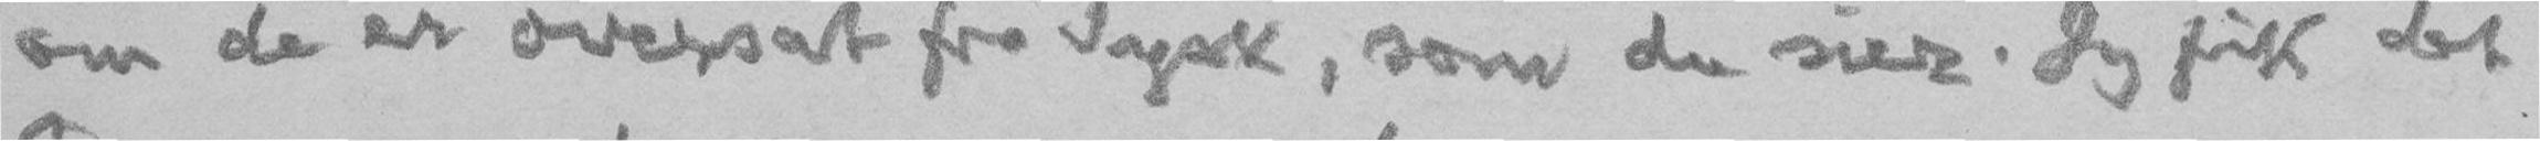om de er oversat fra Tysk, som du sier. Jeg fik det
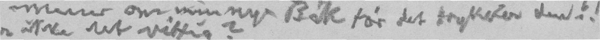mener om min nye Bok før det trykker den!!
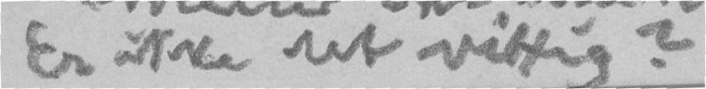Er ikke det vittig?
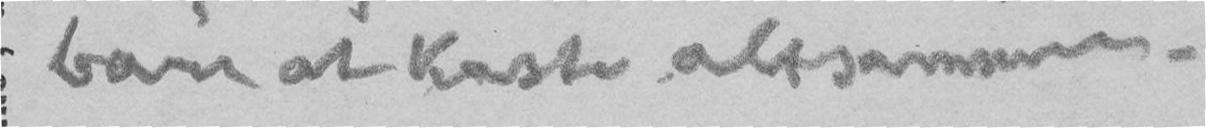bare at kaste altsammen.
